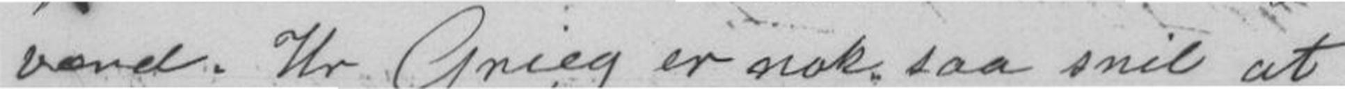værd. Hr Grieg er nok saa snil at
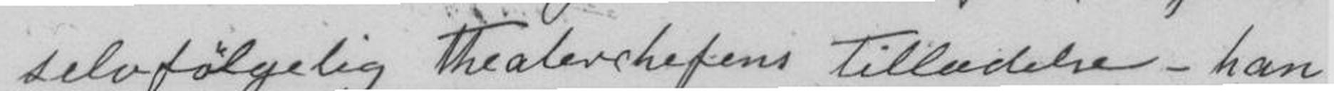selvfølgelig Theaterchefens tillatelse-han
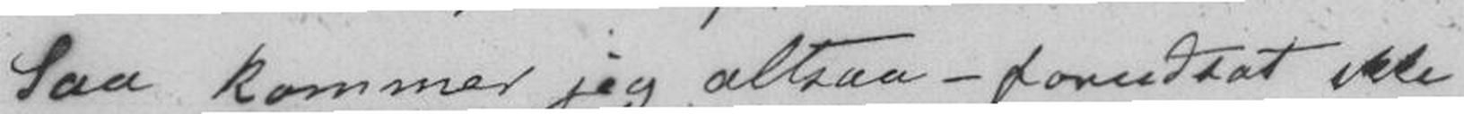Saa kommer jeg altsaa-forudsat ikke
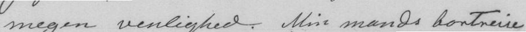megen venlighed. Min mands bortreise
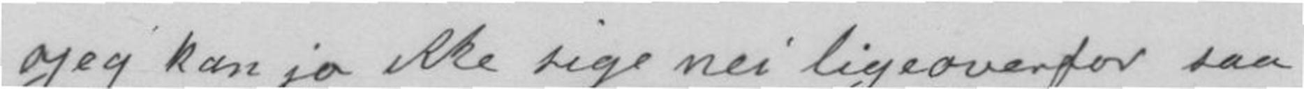Jeg kan jo ikke sige nei ligeoverfor saa
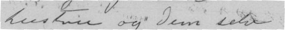hustru og Dem selv
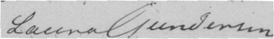Laura Gundersen
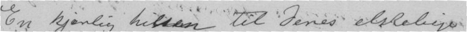En kjærlig hilsen til Deres elskelige
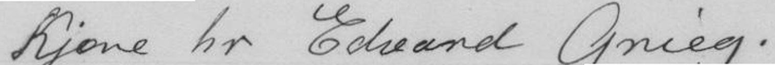Kjære hr Edvard Grieg.
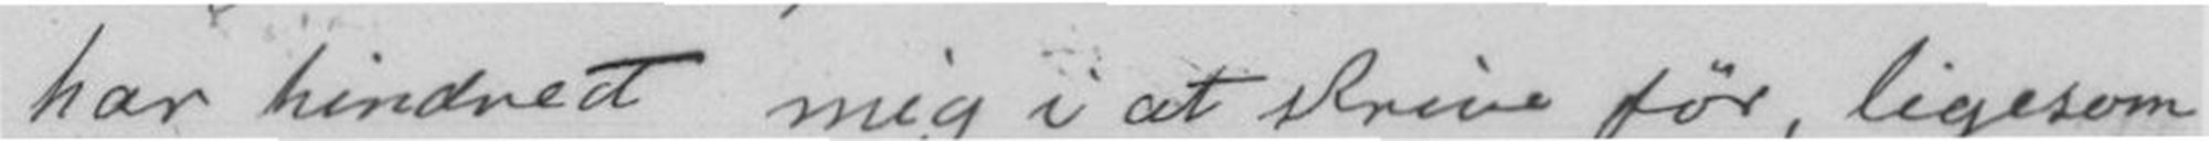har hindret mig i at skrive før, ligesom
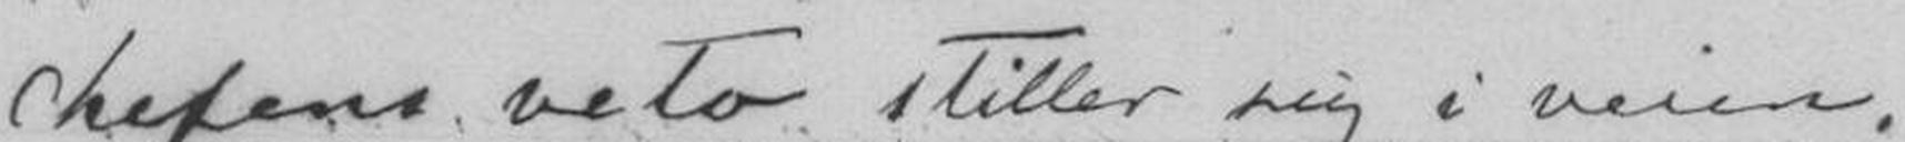chefens veto stiller sig i veien.
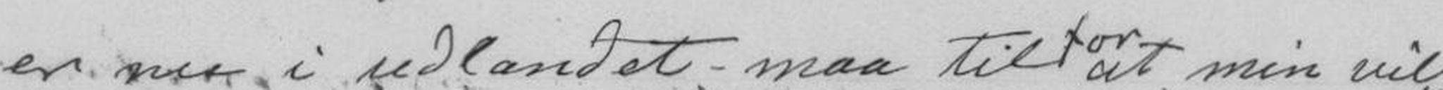er naa i udlandet - maa til for at min vil-
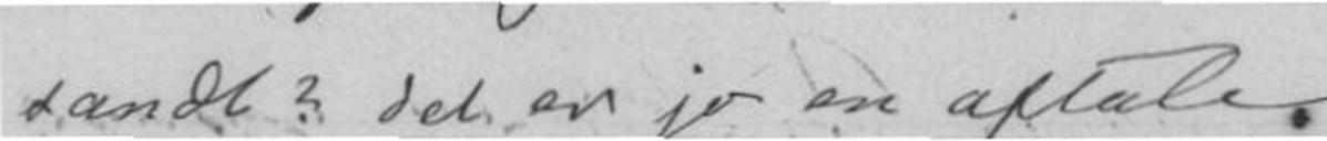sandt? det er jo en aftale.
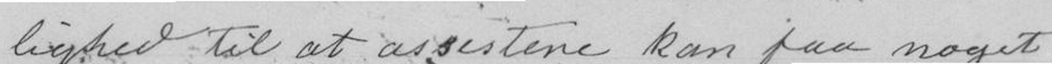ighed til at assistere kan faa noget
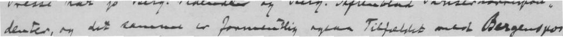denter, og det samme er formentlig ogsaa Tilfældet med Bergenspos-
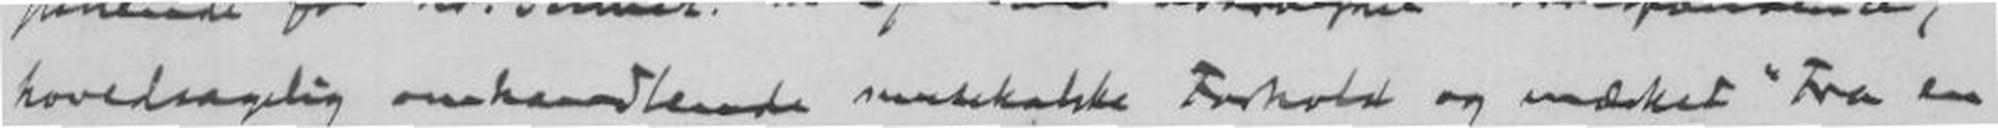hovedsagelig omhandlende musikalske Forhold og mærket "Fra en
